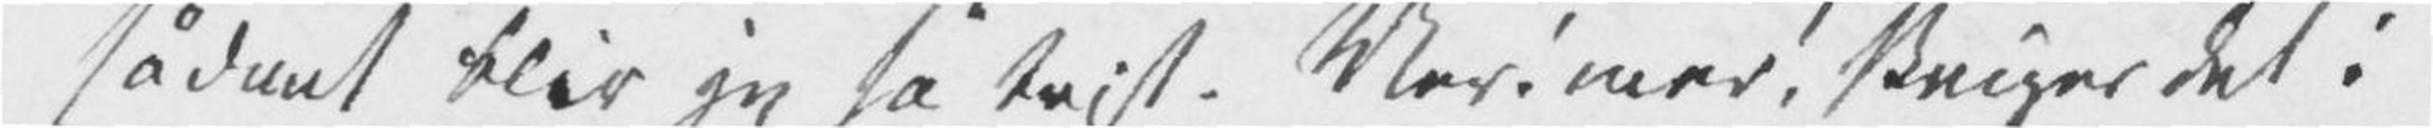sådant blir jeg så trist. Mer! mer! skriger det i
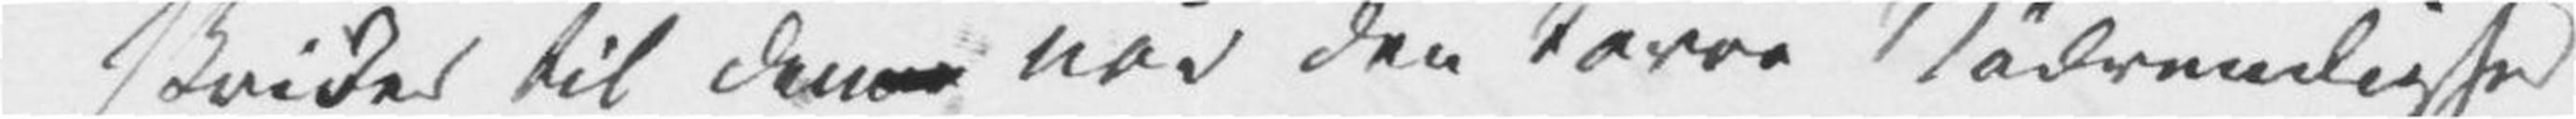skrider til den når den tørre Nødvendighed
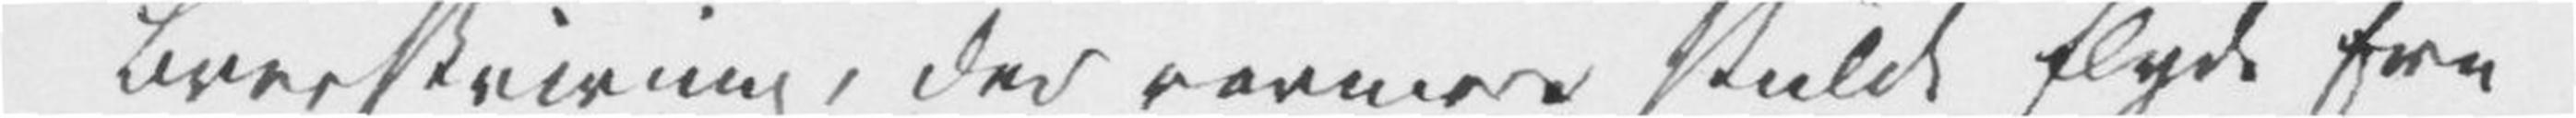Brevskrivning, der varmere skulde flyde fra
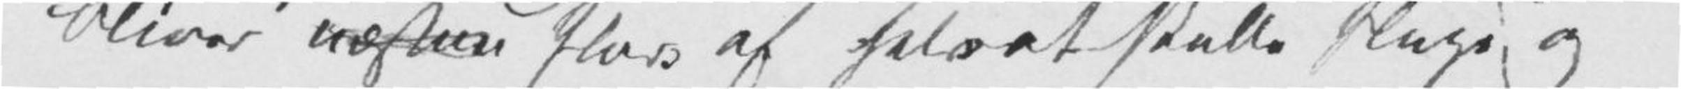bliver næsten flåes af selv at skulle sluge og
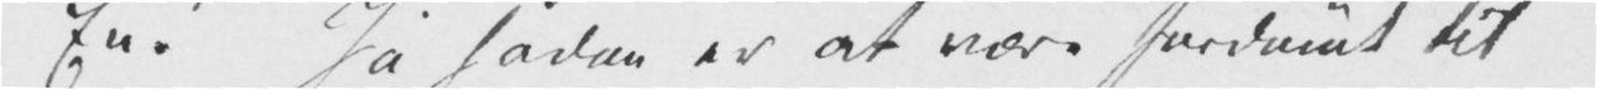En! Ja sådan er [det] at være fordømt til
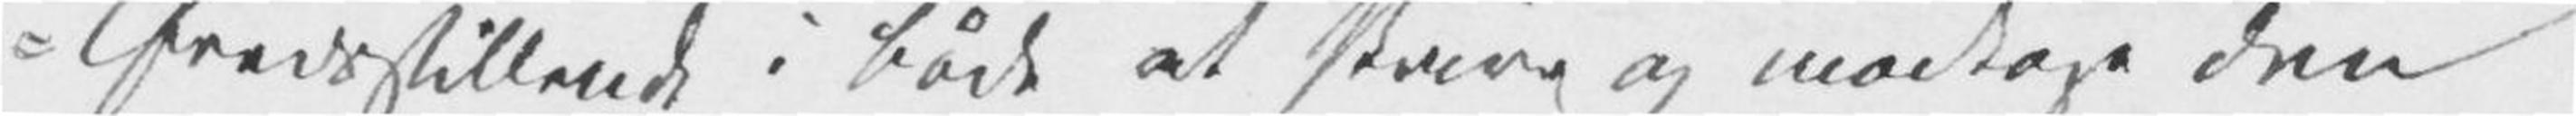fredsstillende i både at skrive og modtage den
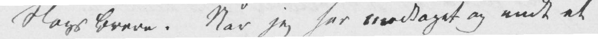Slags Breve. Når jeg har modtaget og endt et
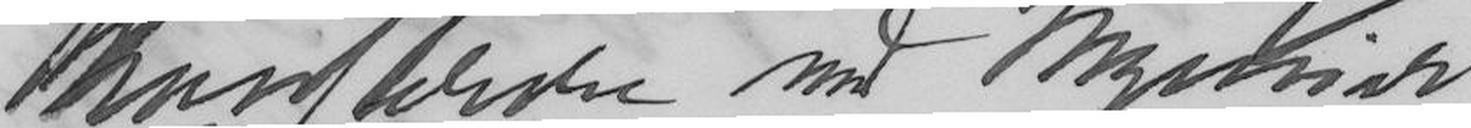comferere med Ingeniør
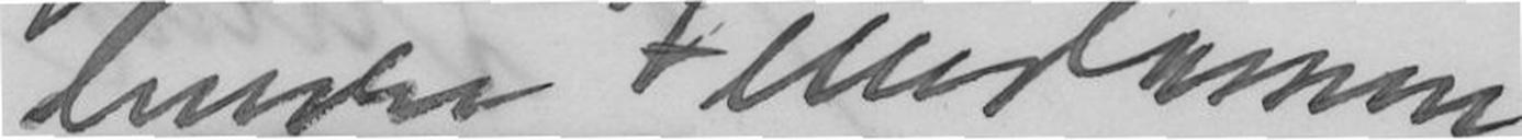levere Fundamen-
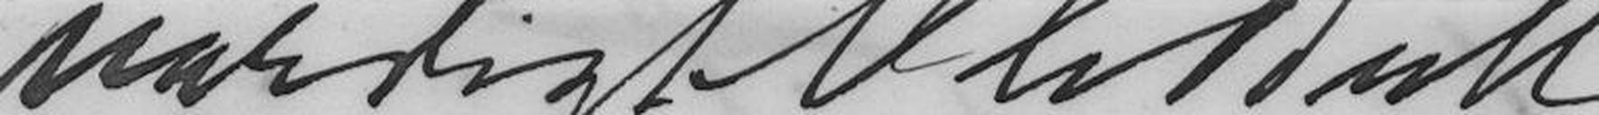værdigt Ole Bull.
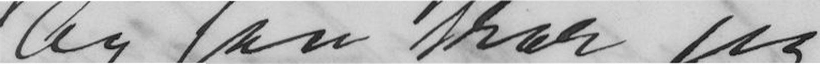Og saa tror jeg
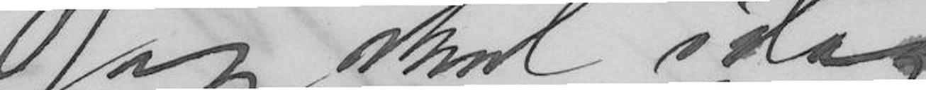Jeg skal idag,
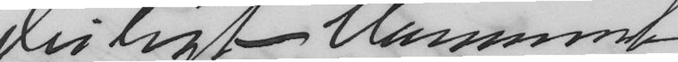deiligt Monument,
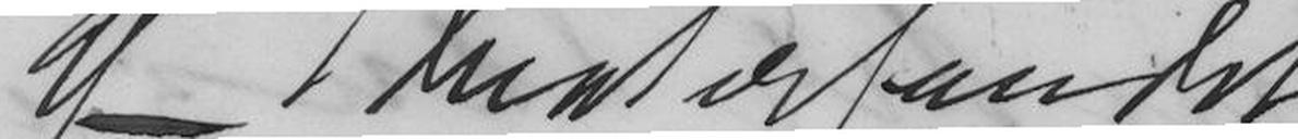af Theaterfondet.
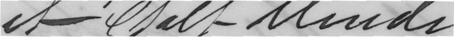et stolt Minde
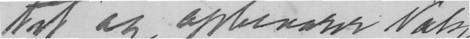tet og opbevarer Nøk-
